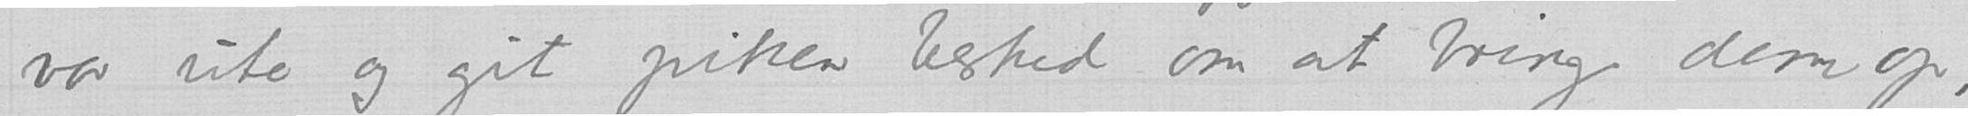var ute og git piken besked om at bringe dem op,
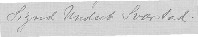Sigrid Undset Svarstad.
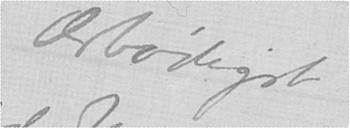Ærbødigst
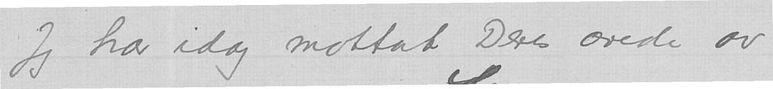Jeg har idag mottat Deres ærede av
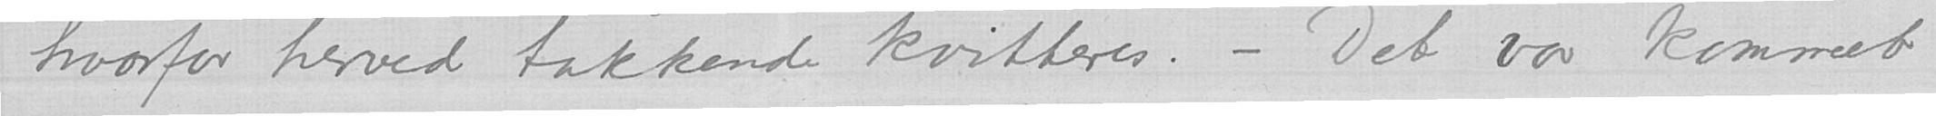hvorfor herved takkende kvitteres. - Det var kommet
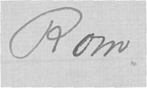Rom
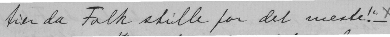tier da Folk stille for det meste! - x
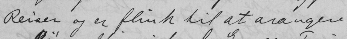Reiser og er flink til at arangere
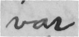var
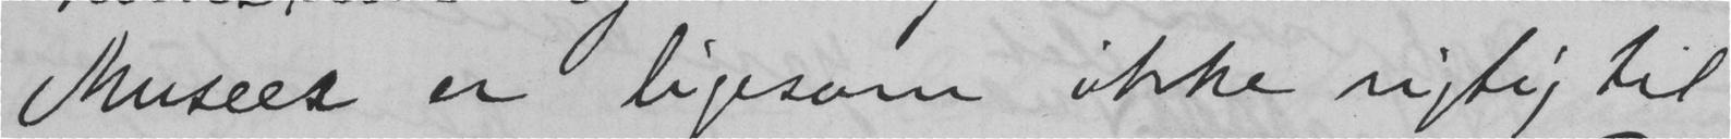museer er ligesom ikke rigtig til
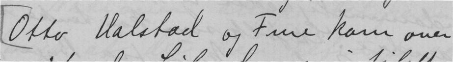Ofto Valstad og Frue kom over
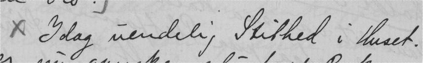x Idag uendelig Stilhed i Huset.
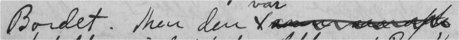Bordet. Men den
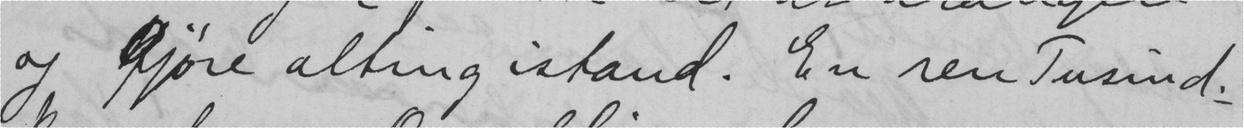og gjøre alting istand. En ren Tusind-
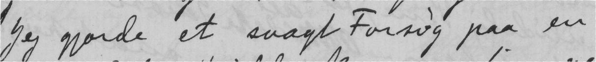Jeg gjorde et svagt Forsøg paa en
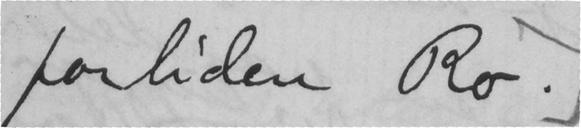forliden Ro.
